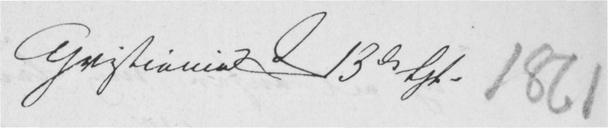Christiania d. 13de Spt.
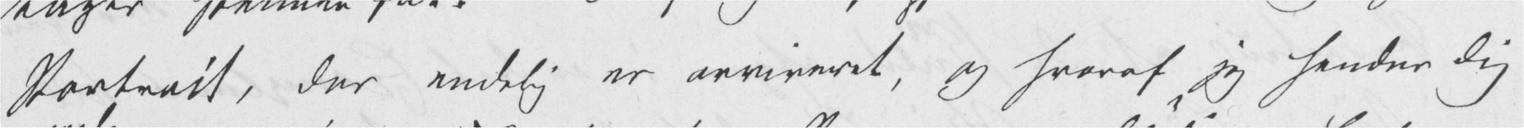Portrait, der endelig er arriveret, og hvoraf jeg sender Dig
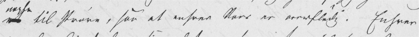et til Prøve, saa at enhver Roes er overflødig. Enhver
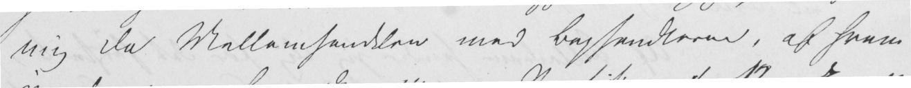mig da Mellemhandelen med Boghandlerne, af hvem
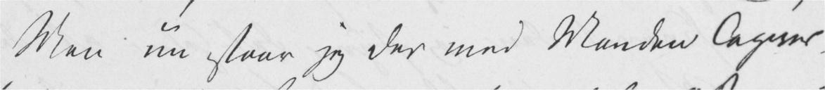Men nu staar jeg der med Manden Tegnér,
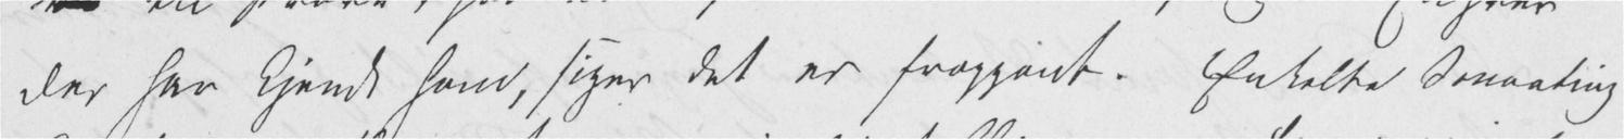der har kjendt ham, siger det er frappant. Enkelte Smaating
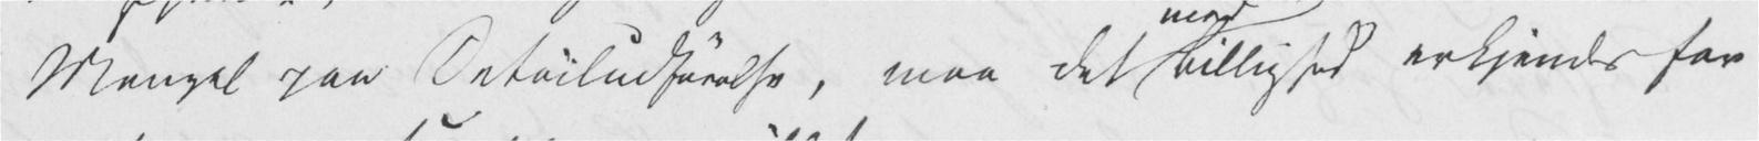Mangel paa Detailudførelse, maa det Billighed erkjendes for
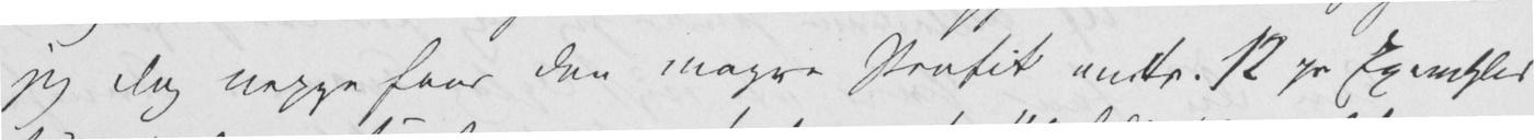jeg dog neppe faar den magre Profit omtr. 12 pr Exemplar
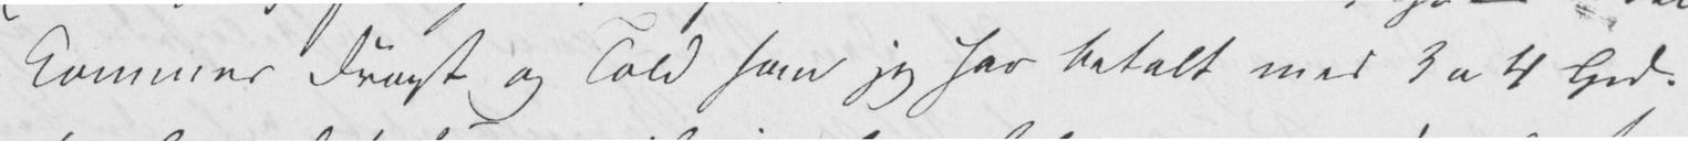kommer Fragt og Told som jeg har betalt med 3 a 4 Spd.
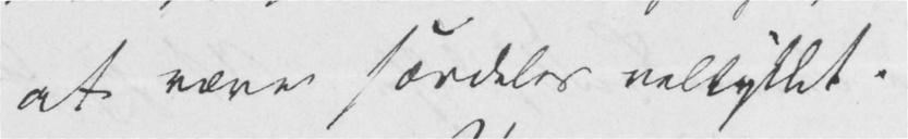at være særdeles vellykket.
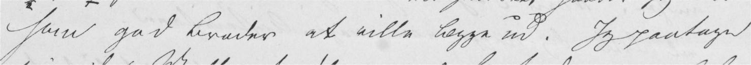som god Broder at ville lægge ud. Jeg paatager
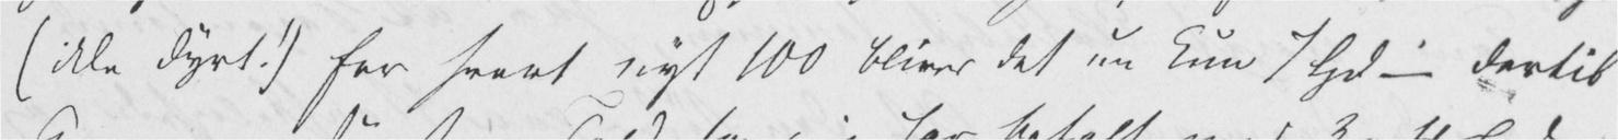(ikke dyrt!) for hvert nyt 100 bliver det nu kun 7 Spd – dertil
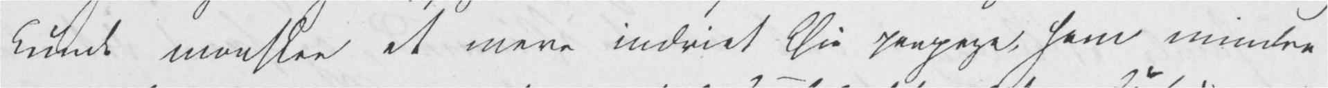kunde maaskee et mere indviet Øie paapege, som mindre
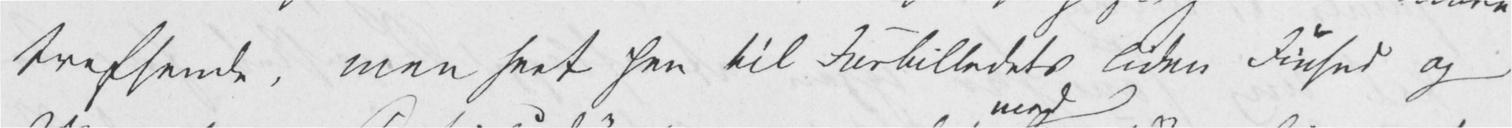treffende, men seet hen til Forbilledets liden Finhed og
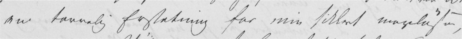en tarvelig Erstatning for min sikkert mageløse,
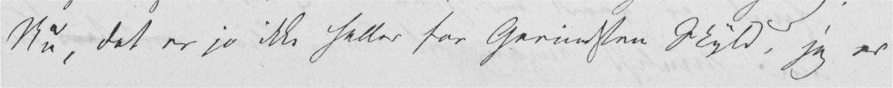Nu, det er jo ikke heller for Gevindsten Skyld, jeg er
